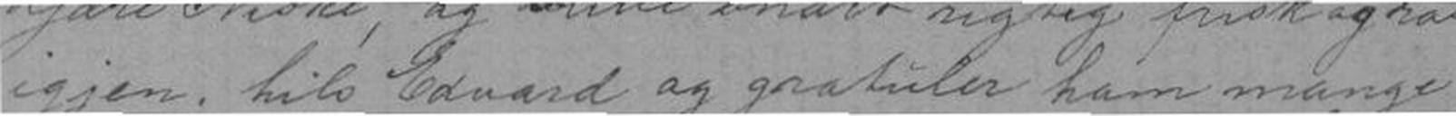igjen; hils Edvard og gratuler ham mange
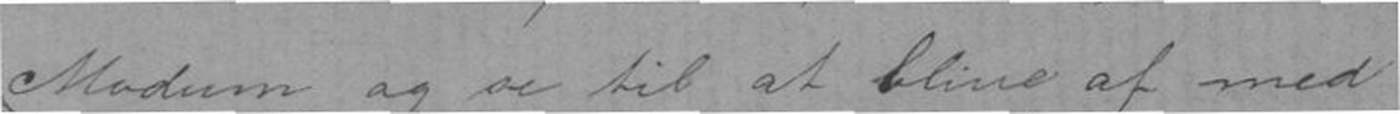Modum og se til at blive af med
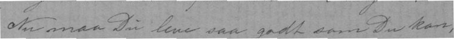Nu maa Du leve saa godt som Du kan,
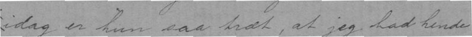idag er hun saa træt, at jeg lod hende
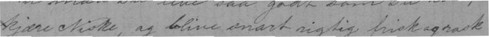kjære Niske, og blive snart rigtig frisk og rask
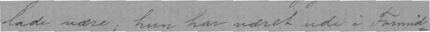lade være; hun har været ude i Formid-
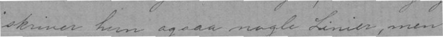skriver hun ogsaa nogle Linier, men
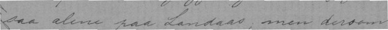saa alene paa Landaas; men dersom
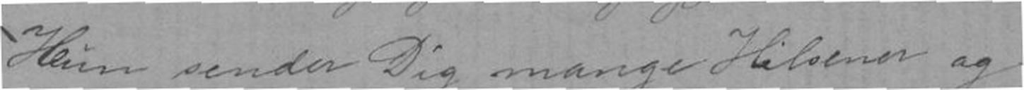Hun sender Dig menge Hilsener og
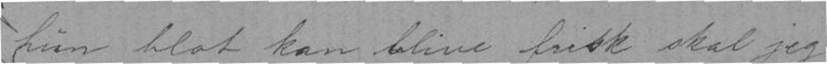hun blit kan blive frisk skal jeg
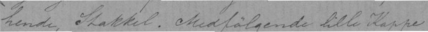hende, Stakkel. Medfølgende lille Kappe
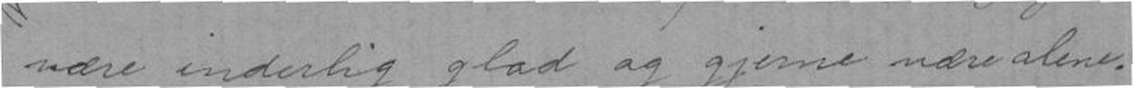være inderlig glad og gjerne være alene.
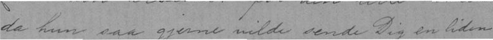da hun saa gjerne vilde sende Dig en liden
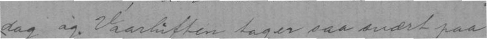dag og Vaarluften tager saa svært paa
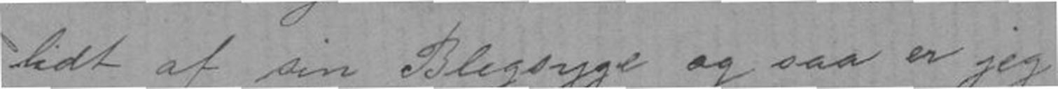lidt af sin Blegsyge og saa er jeg
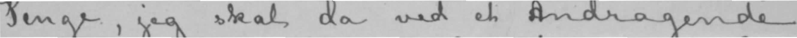Penge, jeg skal da ved et Andragende
